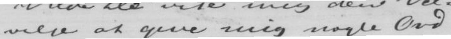vilje at give nogle Ord
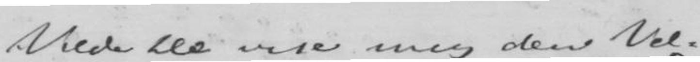Vilde De vise mig den Vel-
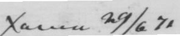Xania 29/6 71
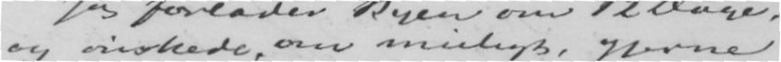og ønskede, om muligt, gjerne
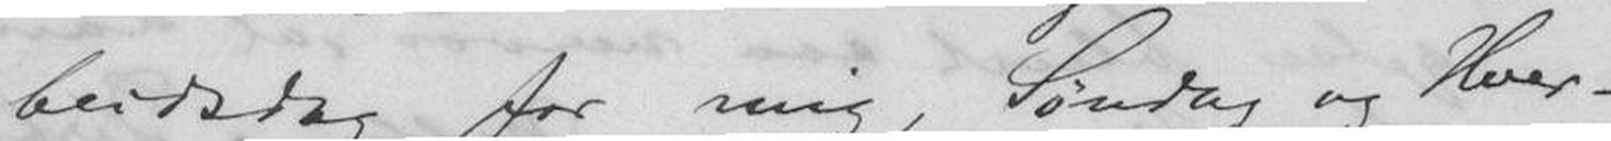beidsdag for mig, Søndag og Hver-
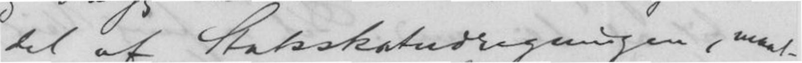del af Statsskatudregningen, maat-
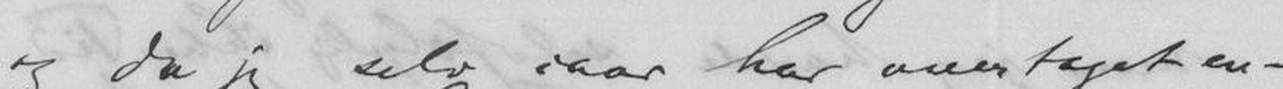og da jeg selv iaar har overtaget en-
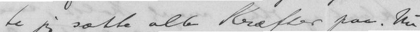te jeg sætte alle Kræfter paa. Nu
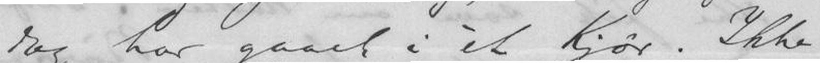dag har gaaet i èt Kjør. Ikke
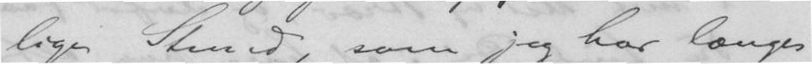lige Stund, som jeg har længes
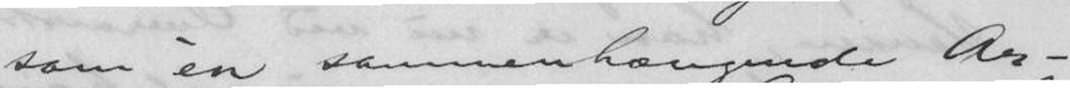som èn sammenhængende Ar-
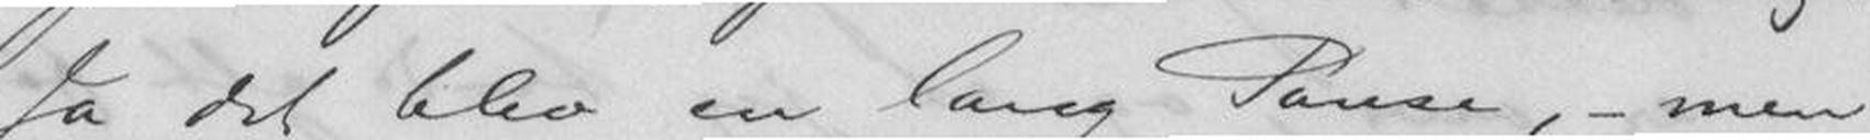Ja det blev en lang Pause, - men
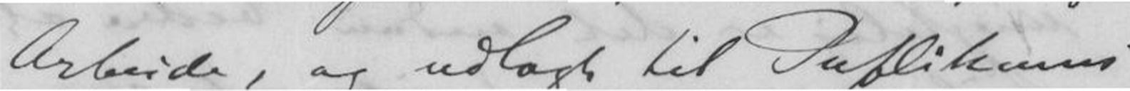Arbeide, og udlagt til Publikums
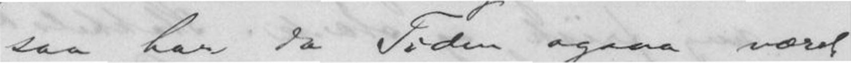saa har da Tiden ogsaa været
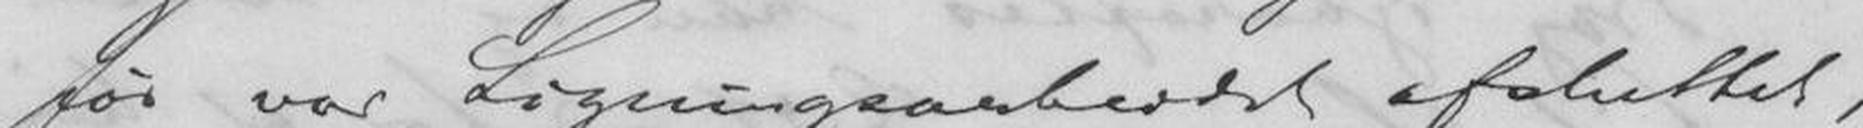før var Ligningsarbeidet afsluttet,
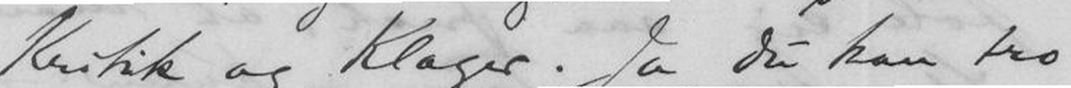Kritik og Klager. Ja, du kan tro
